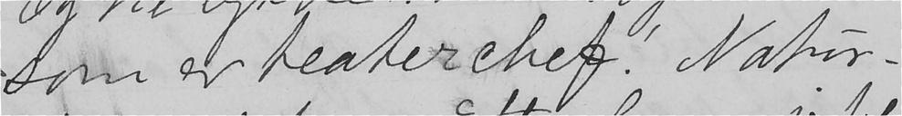som er teaterchef! Natur-
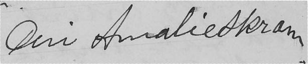Din Amalie Skram.
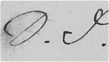D.S.
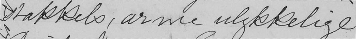stakkels, arme ulykkelige
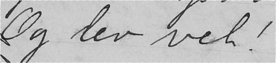Og lev vel!
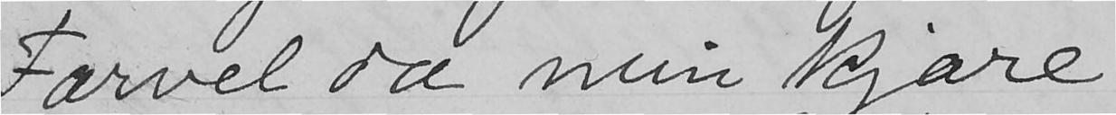Farvel da min kjære
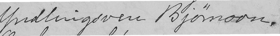yndlingsven Bjørnson.
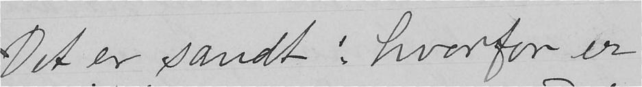Det er sandt: hvorfor er
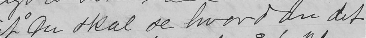Å Du skal se hvordan det
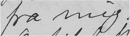fra mig.
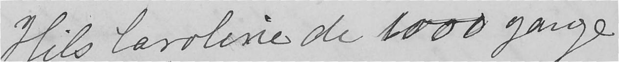Hils Karoline de 1000 gange
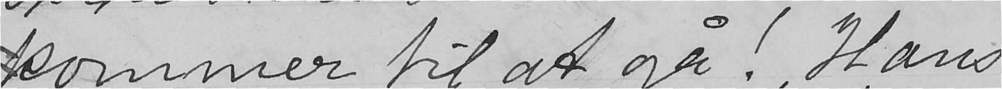kommer til at gå! Hans
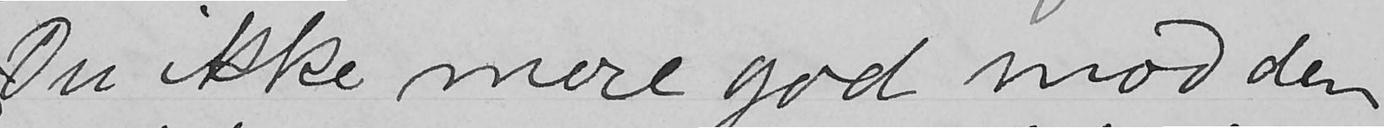Du ikke mere god mod den
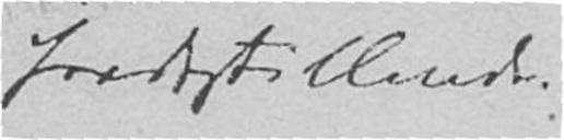fredsstillende.
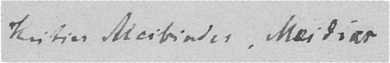trities Ricibioder, elleitiar
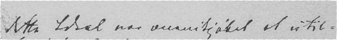dette Ideal var ovenikjøbet et util-
